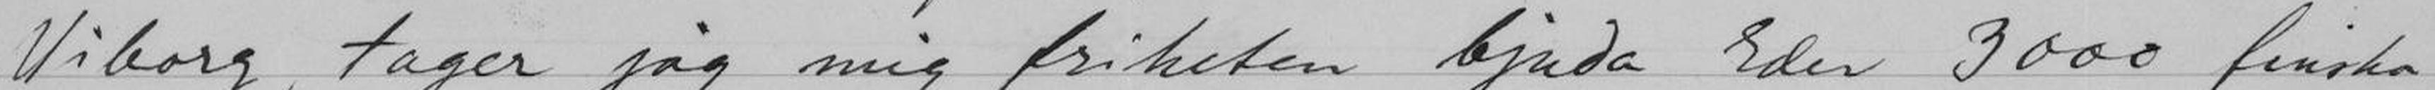Viborg, tager jag mig friheten bjuda Eder 3000 finska
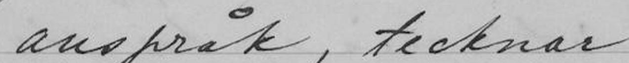anspråk, tecknar
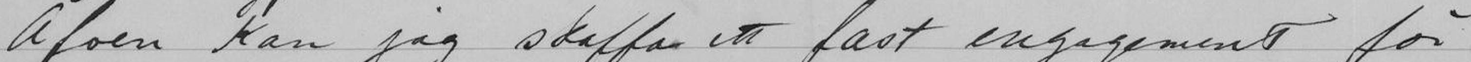Äfven kan jag skaffa ett fast engagement för
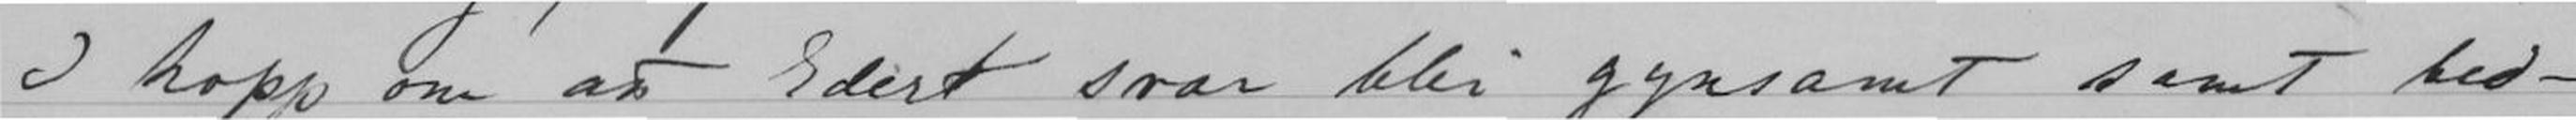I hopp om att Edert svar blir gynsamt samt bed-
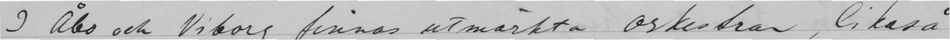I Åbo och Viborg finnas utmärkta orkestrar, likaså
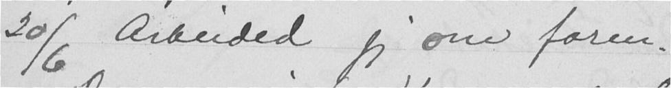20/2 Arbeided jeg om form.
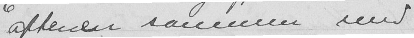aftenen sammen med
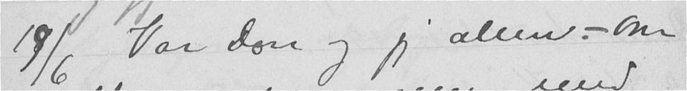19/6 Var Don og jeg alene. - om
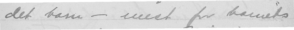det barn - mest for barnets
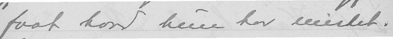faat hvad hun har mistet.
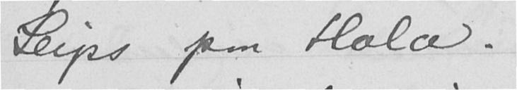Seips paa Hola.
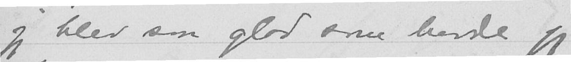jeg blev saa glad som havde jeg
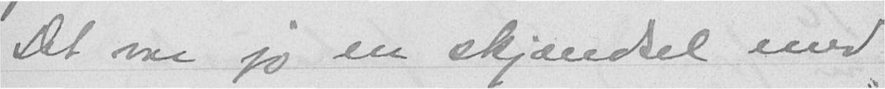Det var jo en skjændsel med
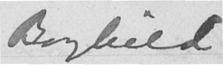Borghild
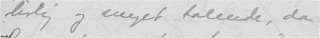livlig og meget talende, da
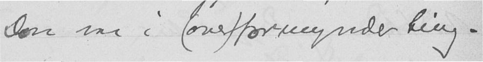Don var i (over)formynderting.
